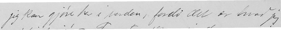jeg kan gjøre her i verden, fordi det er hvad jeg
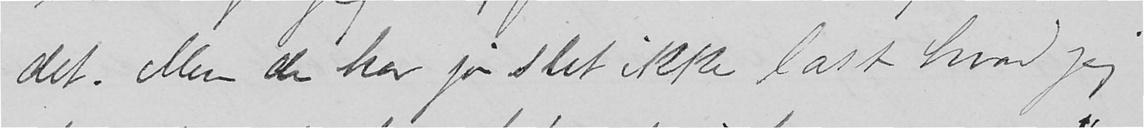det. Men de har jo slet ikke læst hvad jeg
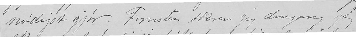nødigst gjør. Forresten skrev jeg dengang jeg
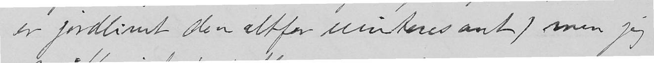er jordlivet der altfor uinteresant) men jeg
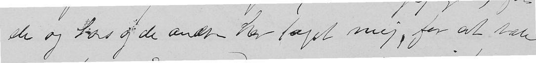de og Sars og de andre har taget mig, for at være
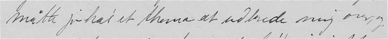måtte jo hae' et thema at udbrede mig over,og
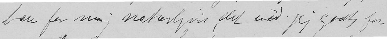bare for mig naturligvis, det ved jeg godt for
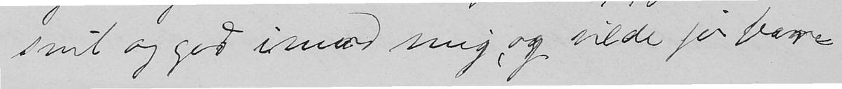snil og god imod mig, og vilde jo bare
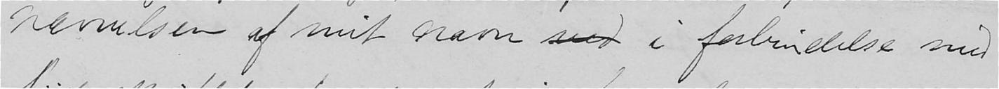nævnelsen af mit navn ved i forbindelse med
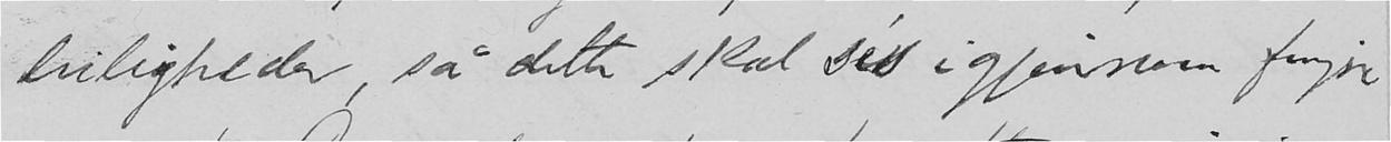leiligheder, så dette skal sés igjennem fingre
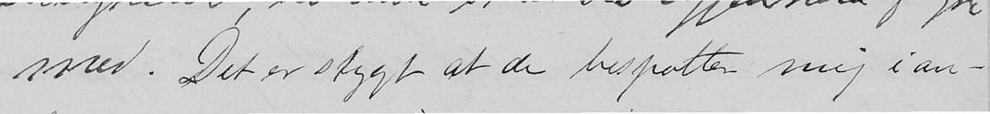med. Det er stygt at de bespotter mig i an-
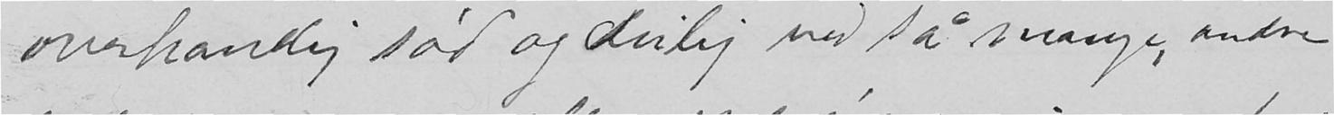ovehændig sød og deilig ved så mange, andre
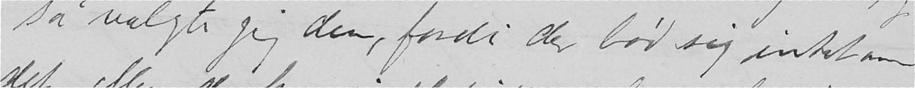så valgte jeg den, fordi der bød sig intet an-
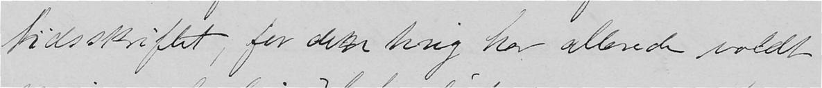tidsskriftet, for den krig har allerede voldt
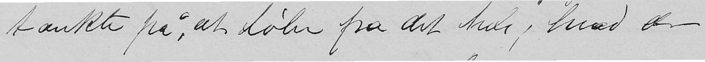tænkte på, at løbe fra det hele, hvad der
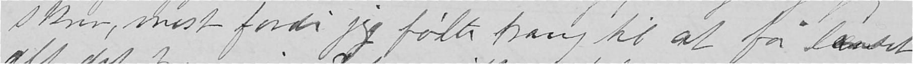skrev, mest fordi jeg følte trang til at få læsset
